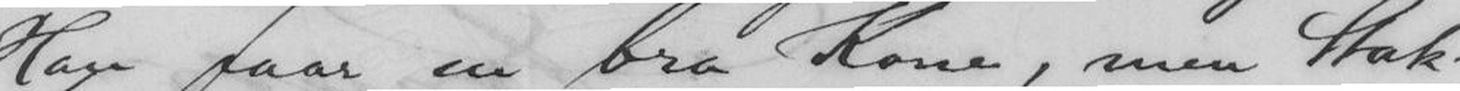Han faar en bra Kone, men Stak-
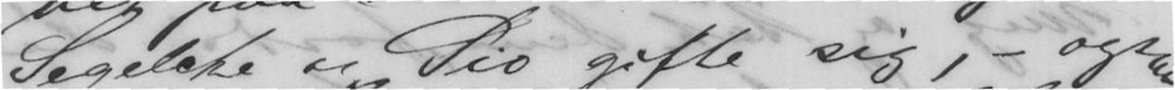Segelcke og Pio gifte sig, - ogsaa
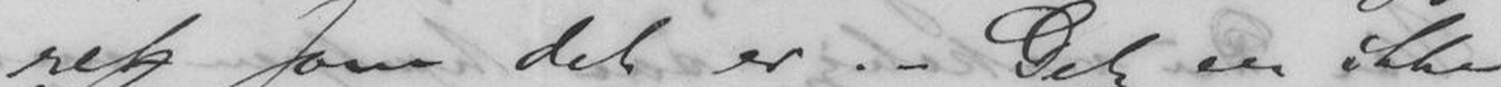ret som det er. - Det er ikke
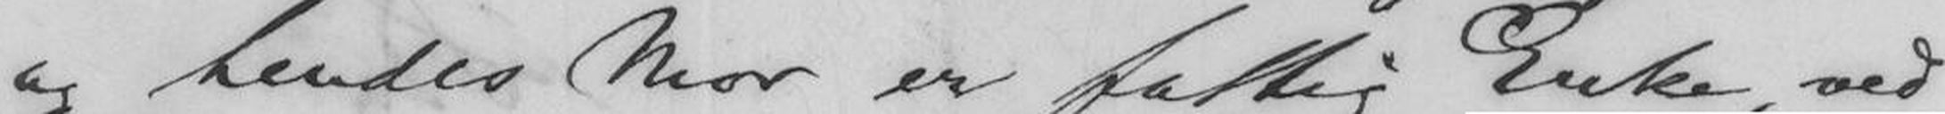og hendes Mor er fattig Enke, ved
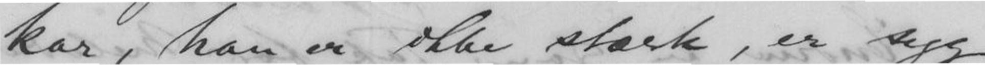kar, han er ikke stærk, er syg
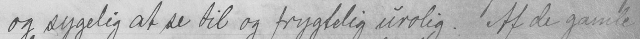og sygelig at se til og frygtelig urolig. Af de gamle
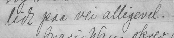lidt paa vei alligevel. -
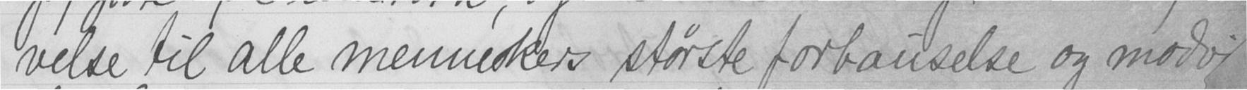velse til alle menneskers største forbanselse og modvi
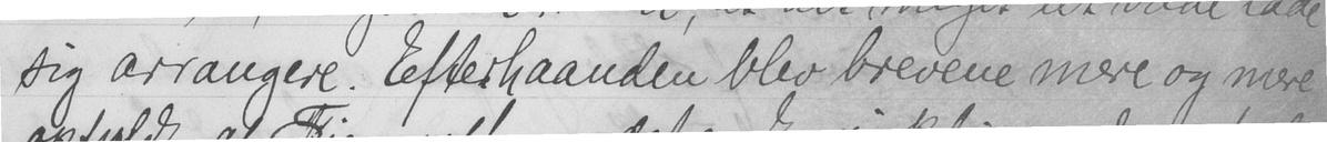sig arrangere. Efterhaanden blev brevene mere og mere
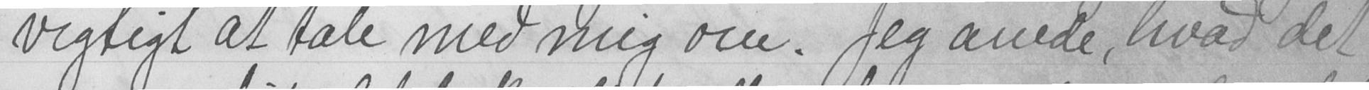vigtigt at tale med mig om. Jeg anede, hvad det
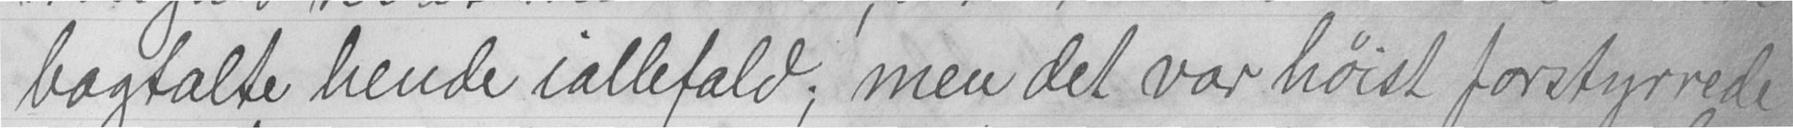bagtalte hende iallefald; men det var høist forstyrrede
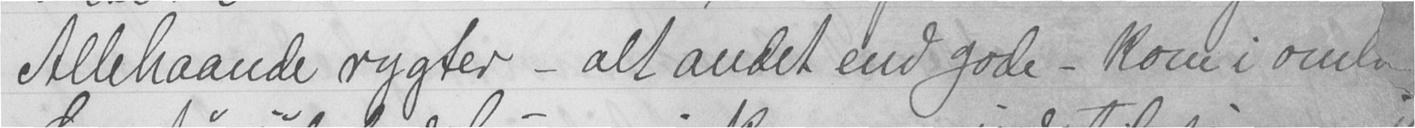Allehaande rygter - alt andet end gode - kom i oml
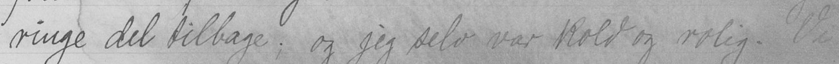ringe del tilbage; og jeg selv var kold og rolig. Vi
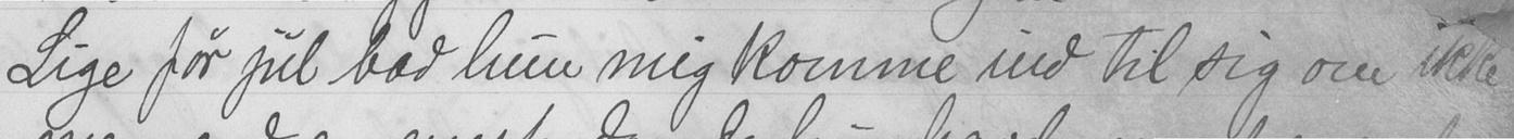Lige før jul bad hun mig komme ind til sig om ikke
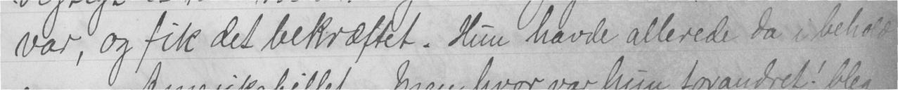var, og fik det bekræftet. Kun havde allerede da i behold
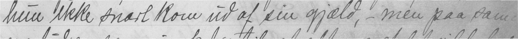hun ikke snart kom ud af sin gjæld, - men paa sam-
